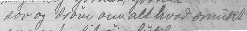sov og drøm om alt hvad smukt
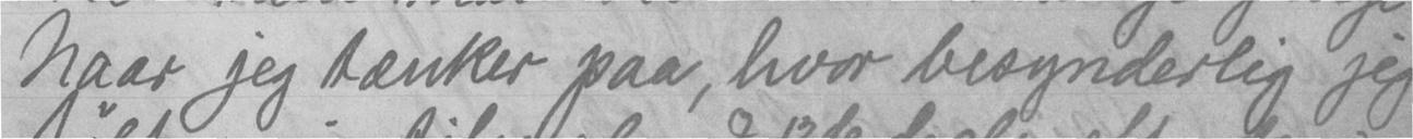Naar jeg tænker paa, hvor besynderlig jeg
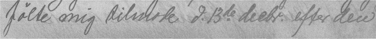følte mig tilmode d. 13de decbr. efter den
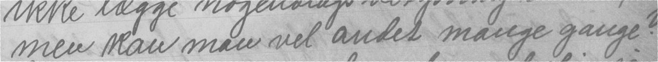men kan man vel andet mange gange?
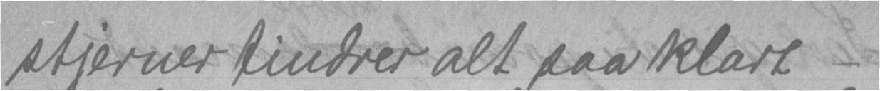stjerner tindrer alt saa klart -
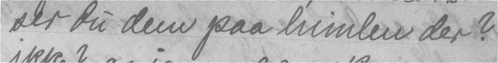ser du dem paa himlen der?
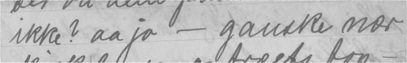ikke? aa jo - ganske nær
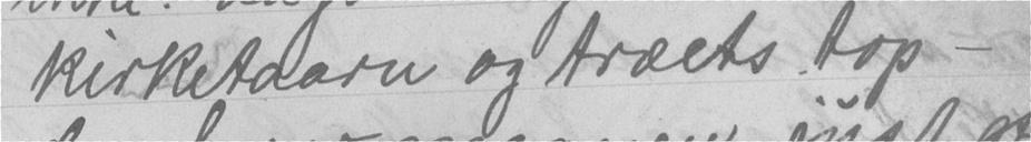kirketaarn og træets top -
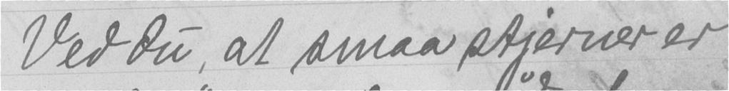Ved du, at smaa stjerner er
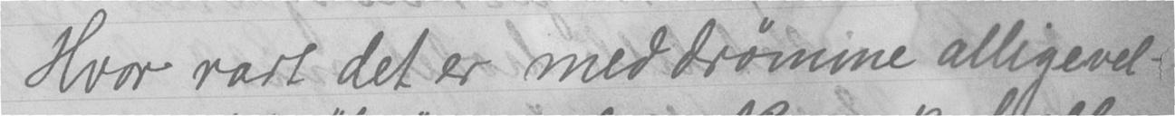Hvor rart det er med drømme alligevel -
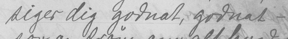siger dig godnat, godnat -
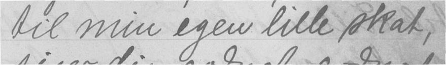til min egen lille skat,
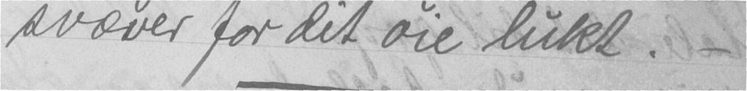svæver for dit øie lukt. -
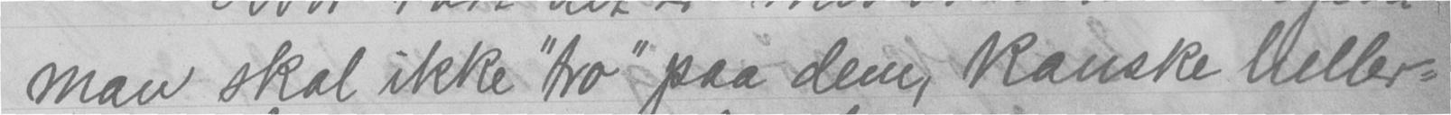man skal ikke "tro" paa dem, kanske heller-
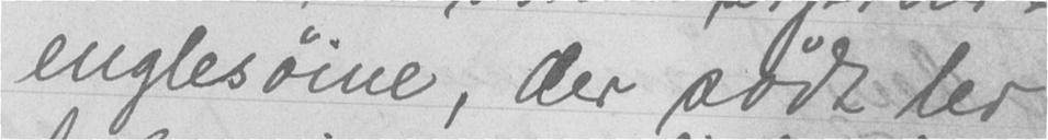engles øine, der sødt ler
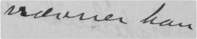næmner han
